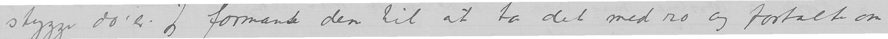stygge do’er. Jeg formante dem til at ta det med ro og fortalte om
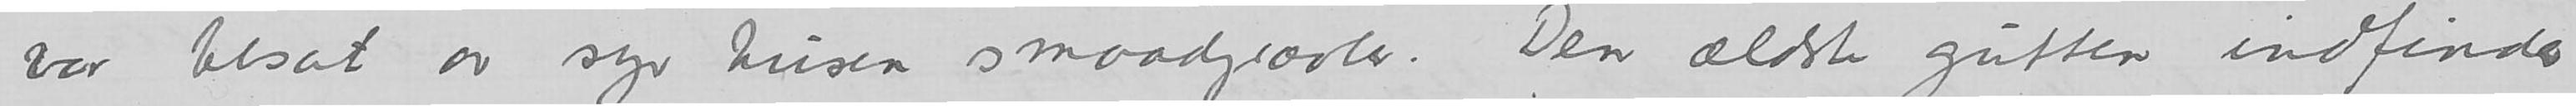var besat av syv tusen smaadjævler. Den ældste gutten indfinder
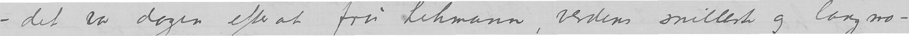– det var dagen efterat fru Lehmann, verdens snilleste og lang-
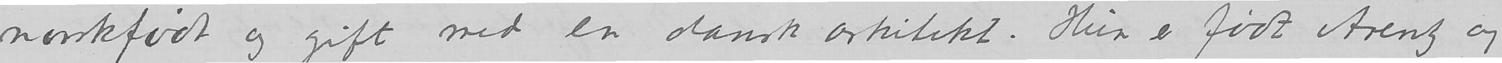– norskfødt og gift med en dansk arkitekt. Hun er født Arenz og
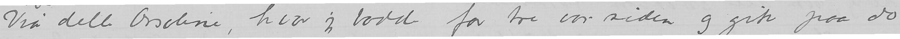Via delle Orsoline, hvor jeg bodde for tre aar siden og gik paa do
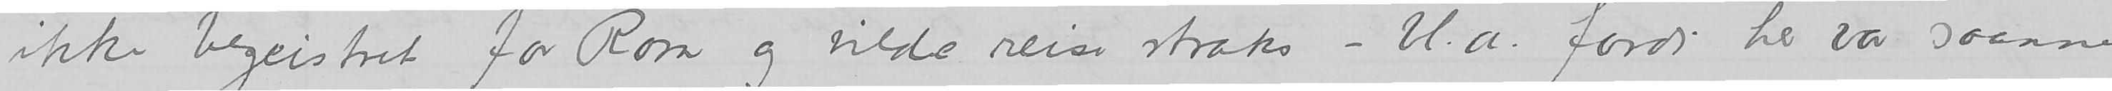ikke begeistret for Rom og vilde reise straks – bl.a. fordi her var saanne
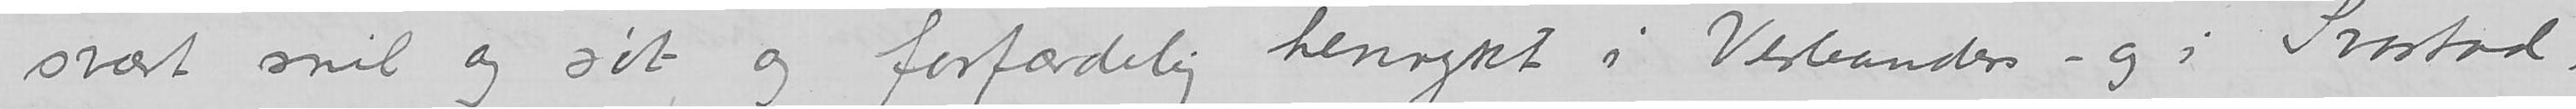svært snil og søt og forfærdelig henrykt i Vesleanders – og i Svarstad,
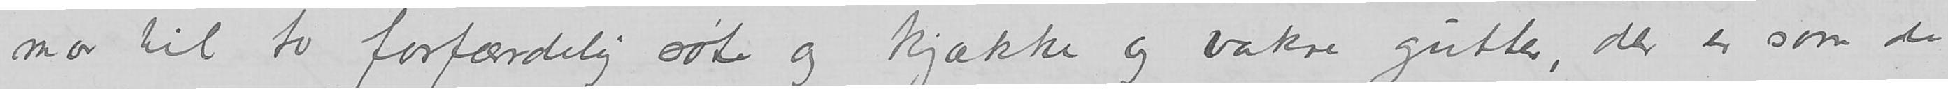mor til to forfærdelig søte og kjække og vakre gutter, der er som de
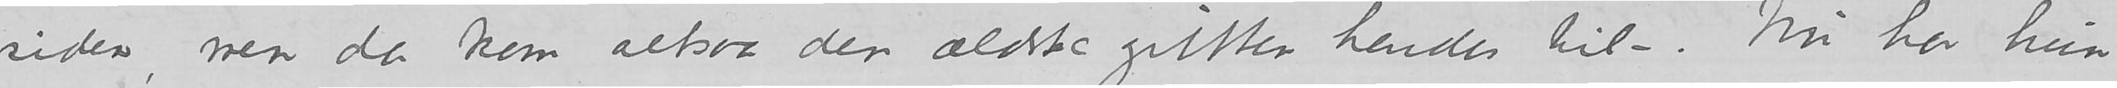siden, men da kom altsaa den ældste gutten hendes til –. Nu har hun
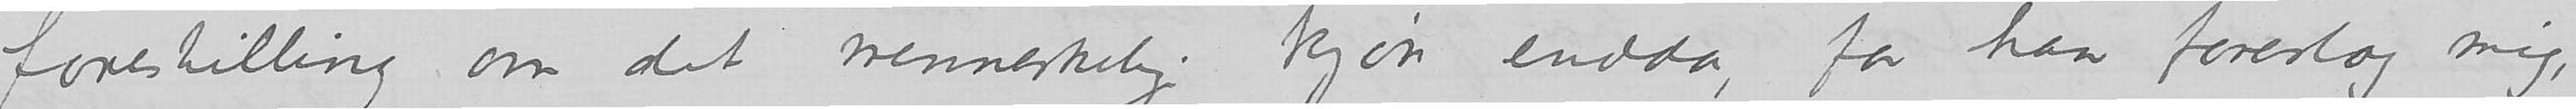forestilling om det menneskelige kjøn endda, for han foreslog mig,
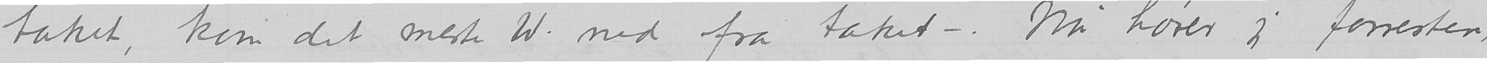taket, kom det meste W. ned fra taket –. Nu hører jeg forresten,
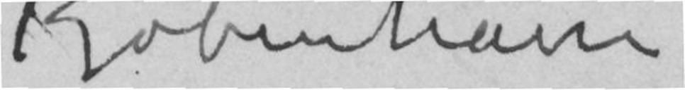Kjøbenhavn
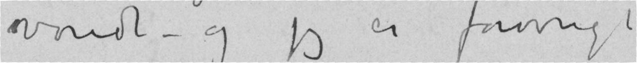vondt – og jeg er forøvrigt
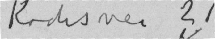Kochsvei 21
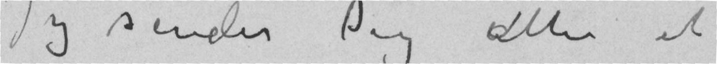Jeg sender dig atter et
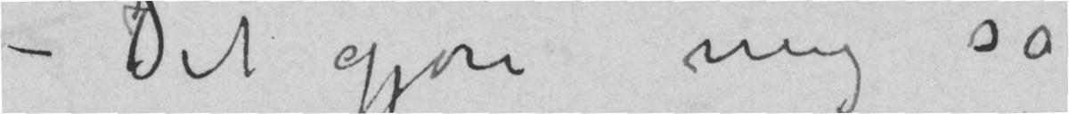– Det gjør mig så
